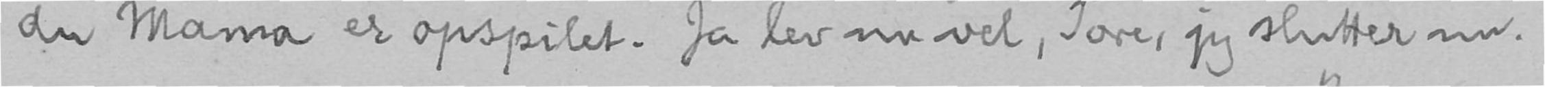du Mama er opspilet. Ja lev nu vel, Tore, jeg slutter nu.
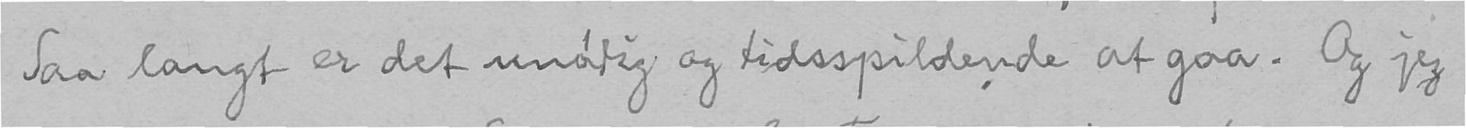Saa langt er det unødig og tidsspildende at gaa. Og jeg
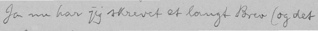Ja nu har jeg skrevet et langt Brev (og det
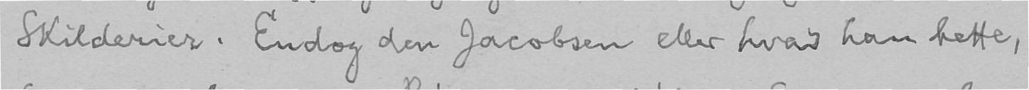Skilderier. Endog den Jacobsen eller hvad han hette,
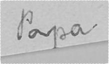Papa
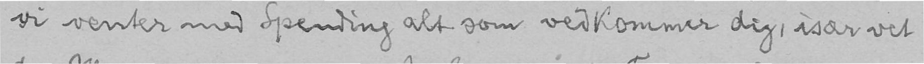vi venter med Spending alt som vedkommer dig, især vet
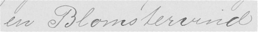en Blomstervind
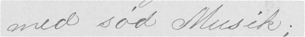med sød Musik;
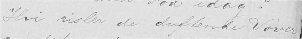Hvi risler de duftende Vover
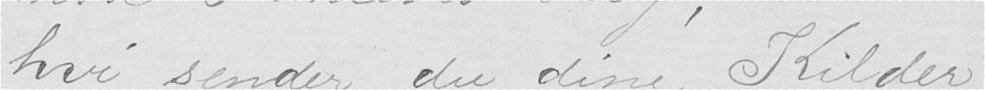hvi sender du dine Kilder
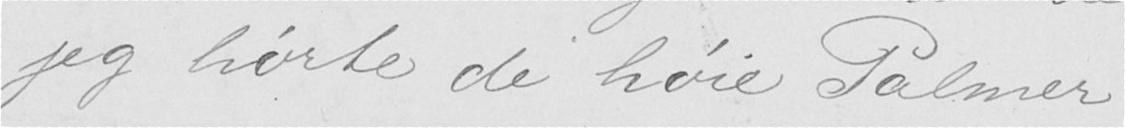jeg hørte de høie Palmer
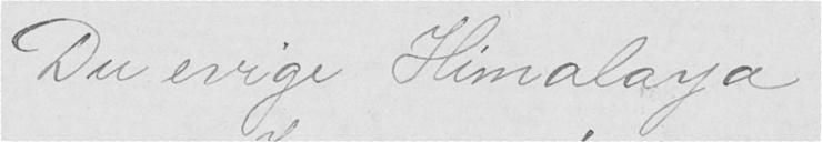Du evige Himalaya
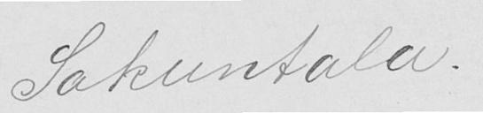Sakuntala.
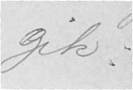gik:
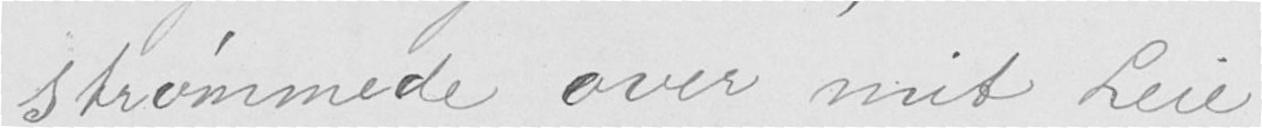strømmede over mit Leie
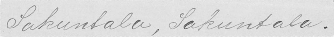Sakuntala, Sakuntala.
